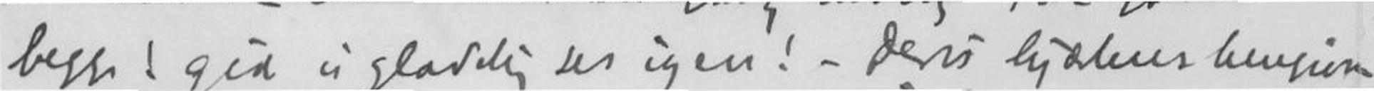begge! gid vi gladelig ses igen! - Deres hjærtens hengivne
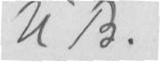U.B.
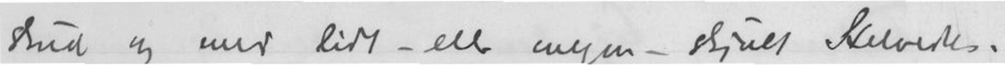skud og med lidt - eller ingen - skjult Helvedes-
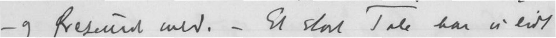- og Øresund med. - Et stort Tale har vi lidt
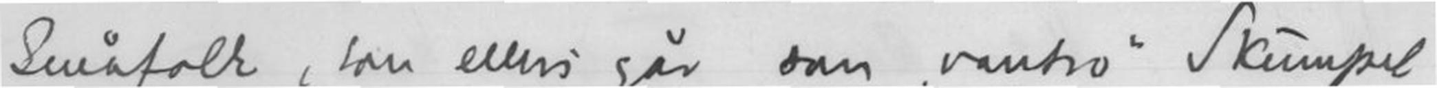Småfolk, som ellers går som vantro Skumpet
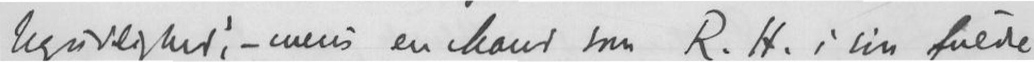Ugudelighed, - mens en Mand som R.H. i sin fulde
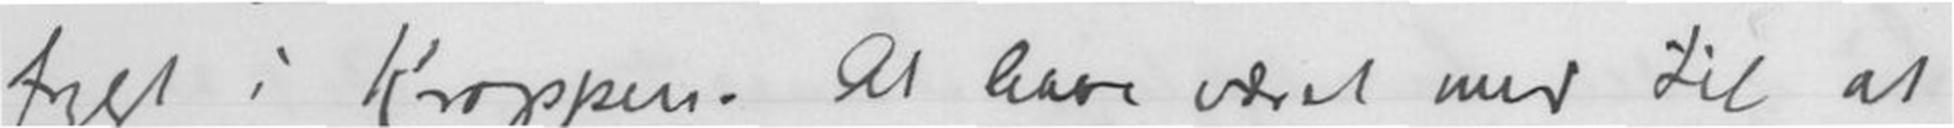fyld i Kroppen. At have været med til at
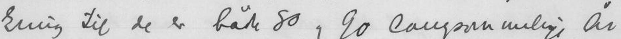kring til de er både 80 og 90 langsommelige År
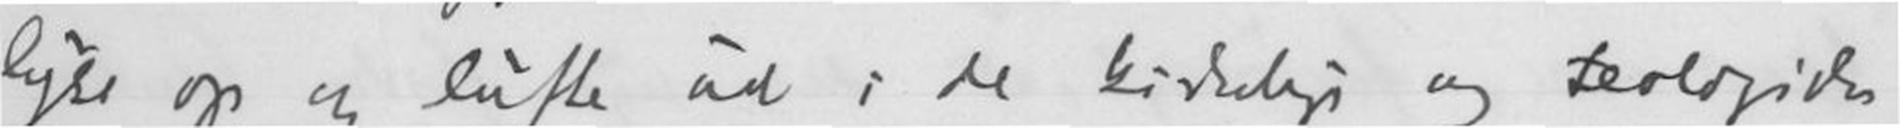lyse op og lufte ud i de kirkelige og teologiske
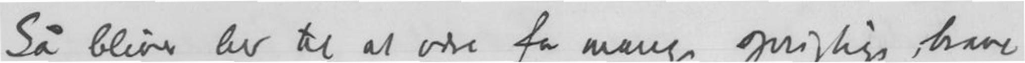Så blive liv til at være for mange oprigtige, brave
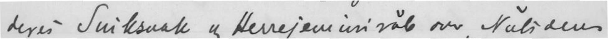deres Sniksnak og Herrejemini råb over Nutidens
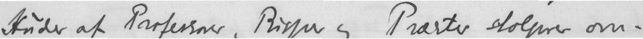Huder af Professorer, Bisper og Præster stolprer om-
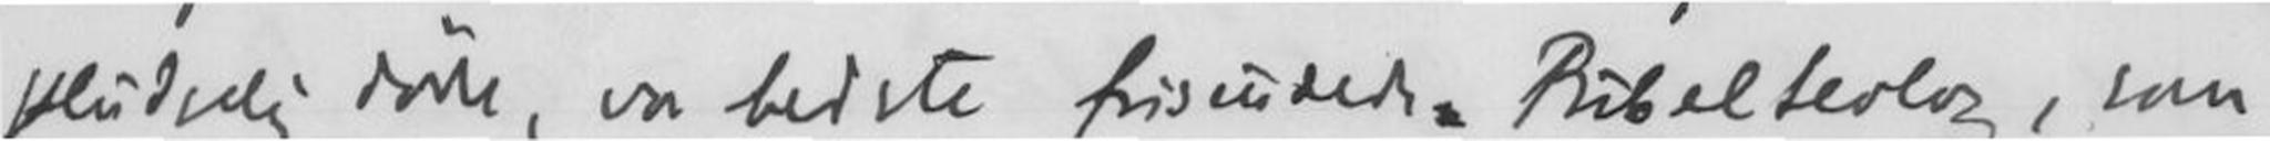pludselig døde, vor bedste frisindede Bibelteolog, som
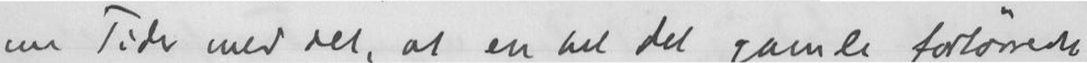me Tide med det, at en hel del gamle forstørrede
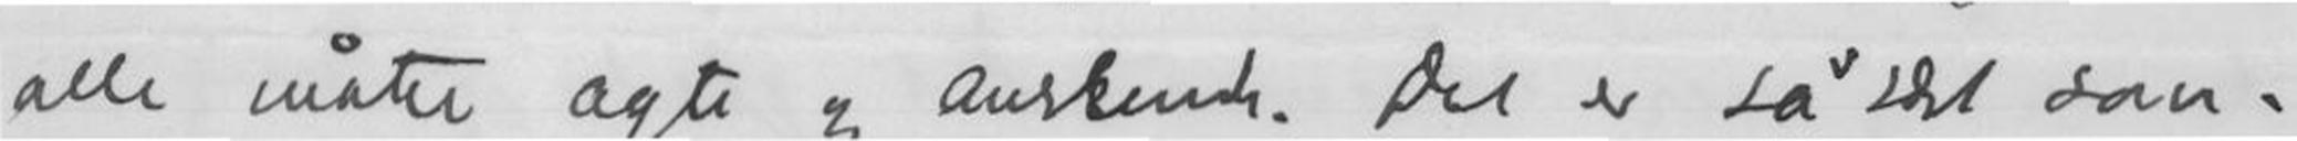alle måtte agte og anskende. Det er så idet sam-
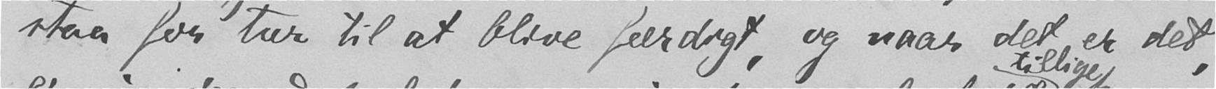staa for tur til at blive færdigt, og naar det er det,
